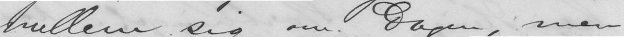mellem sig om Dagen, men
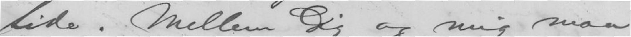tide. Mellem Dig og mig maa
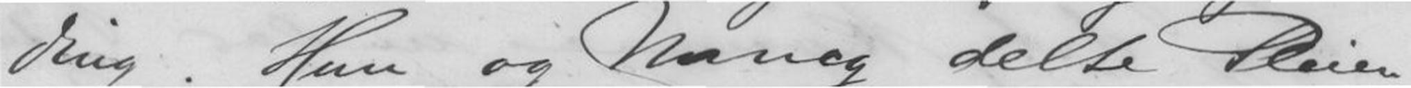ding. Hun og Nancy delte Pleien
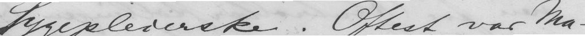Sygepleierske. Oftest var Ma-
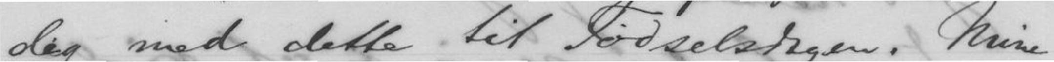dig med dette til Fødselsdagen. Mine
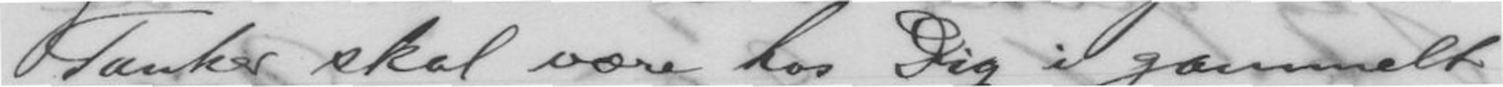Tanker skal være hos Dig i gammelt
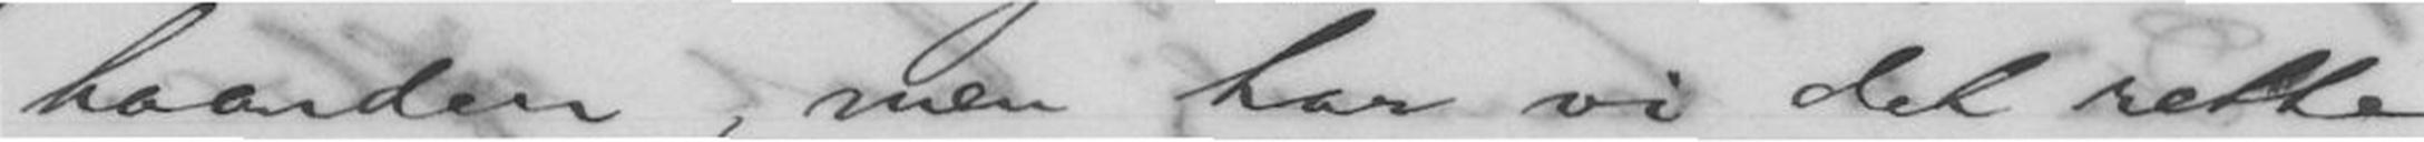haanden, men har vi det rette
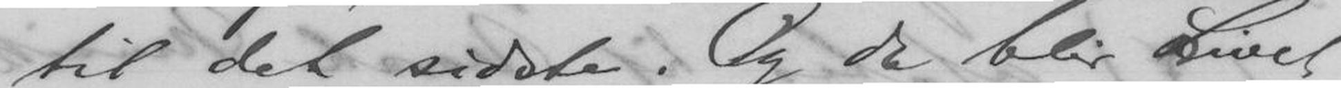til det sidste. Og da blir Livet
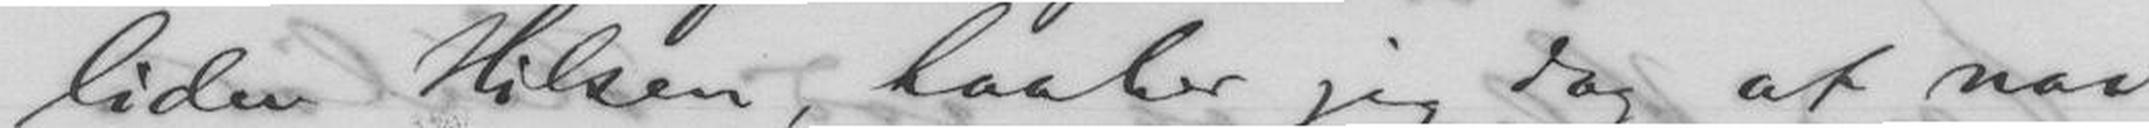liden Hilsen, haaber jeg dog at naa
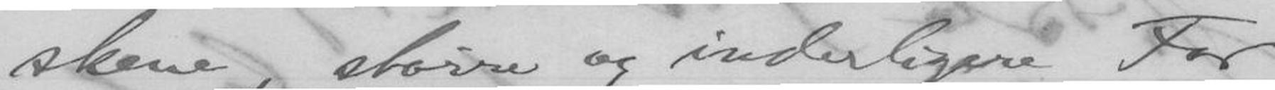skene, større og inderligere For-
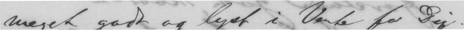meget godt og lyst i Vente for Dig!
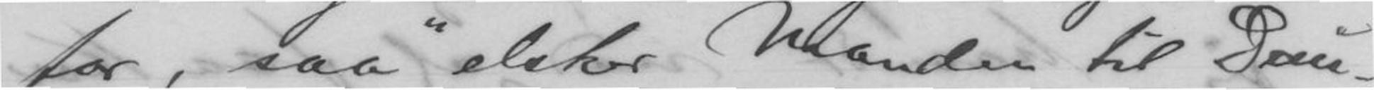for, saa "elsker Manden til Dau-
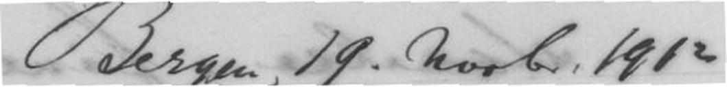Bergen 19. Novbr.1912
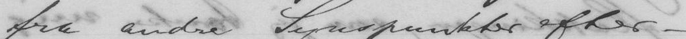fra andre Synspunkter efter-
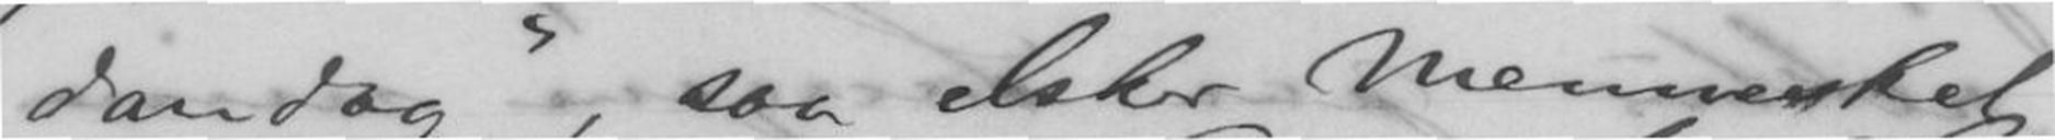dandag", saa elsker Mennesket
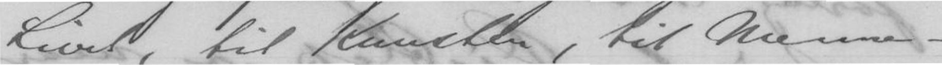Livet, til Kunsten, til Menne-
